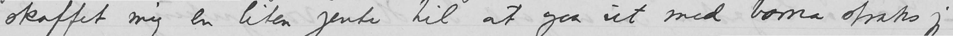skaffet mig en liten jente til at gaa ut med barna straks jeg
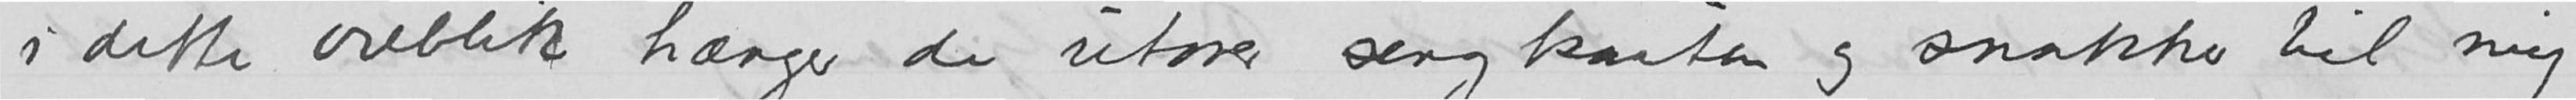i dette øieblik hænger de utover sengkanten og snakker til mig –
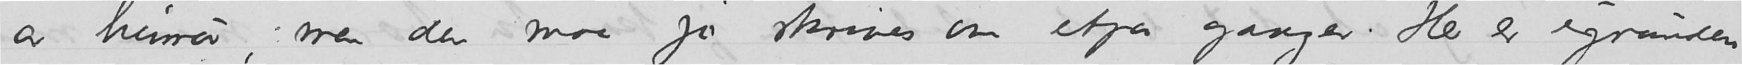av humør, men den maa jo skrives om etpar ganger. Her er igrunden
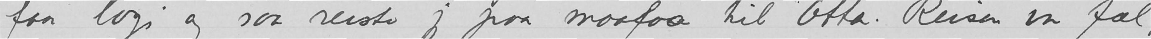faa logi og saa reiste jeg paa maafaa til Otta. Reisen var fæl,
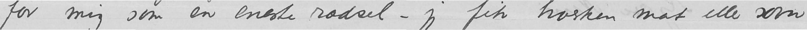for mig som en eneste rædsel – jeg fik hverken mat eller søvn
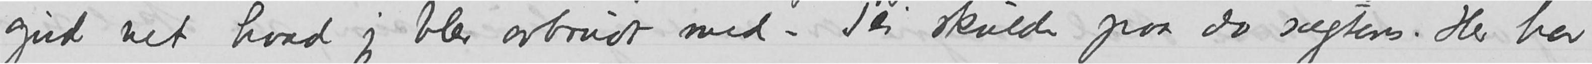gud vet hvad jeg ble avbrudt med - Pei skulde paa do sagtens. Her har
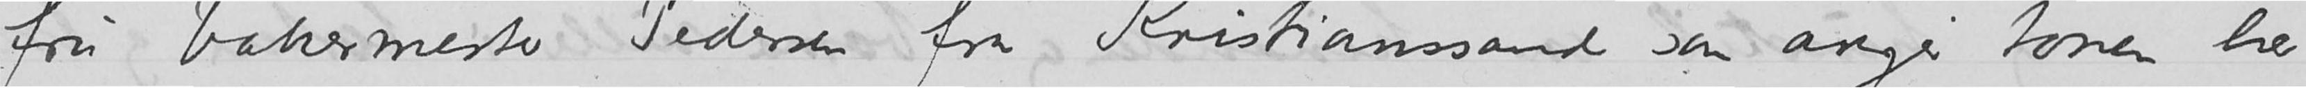fru bakermester Pedersen fra Kristianssand som angir tonen her
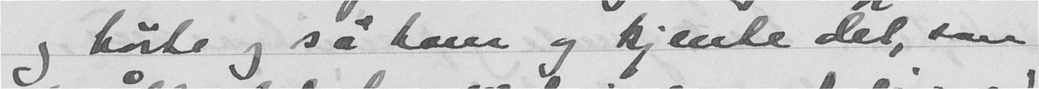og hørte og så ham og kjente det, som
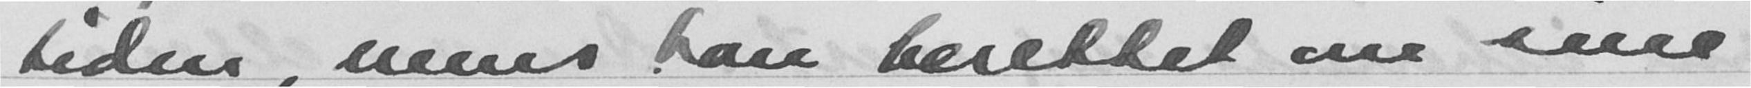tiden, mens han berettet om sine
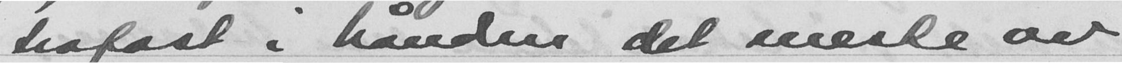trofast i hånden det meste av
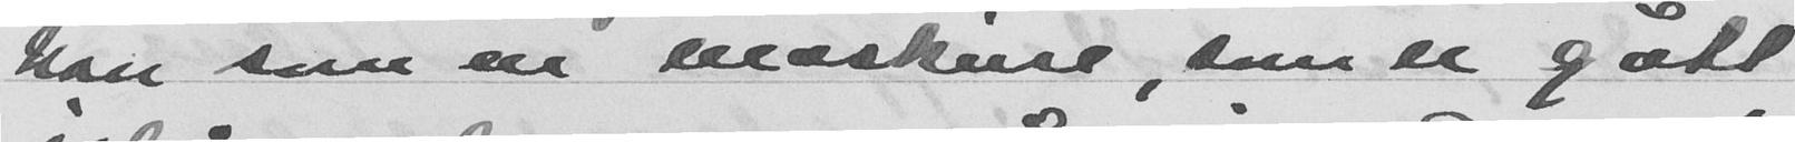han som en maskine, som er gått
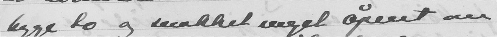begge to og snakket meget åpent om
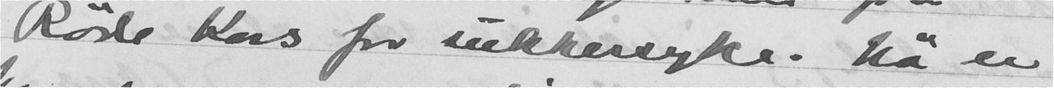Røde Kors for sukkersyke. Nå er
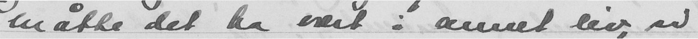måtte det ha vært i annet liv, vi
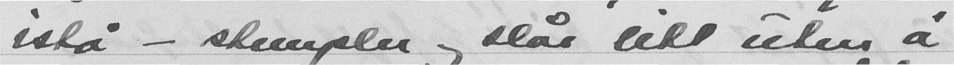istå - stempler og slår litt uten å
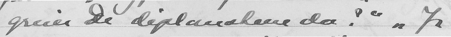greier De diplomatene da?" "Jeg
